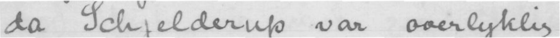da Schjelderup var overlyklig
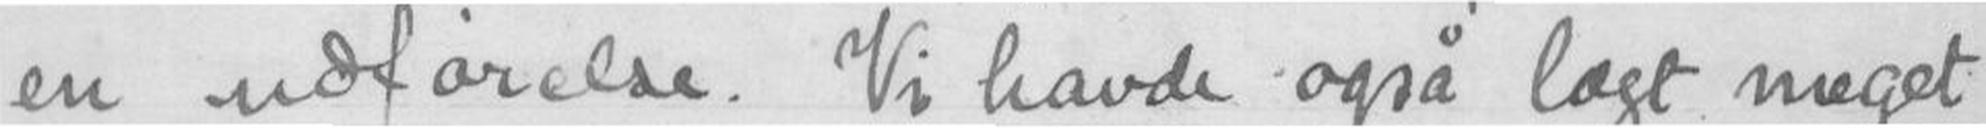en udførelse. Vi havde også lagt meget
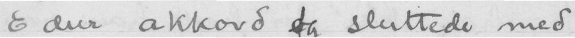E dur akkord og sluttede med
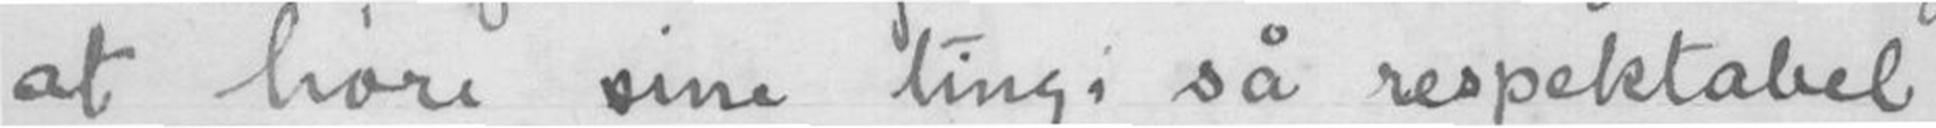at høre sine ting i så respektabel
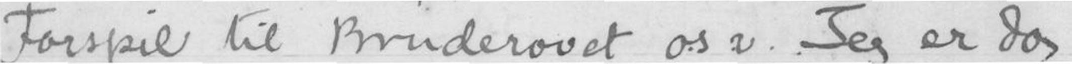Forspil til Bruderovet o.s.v. Jeg er dog
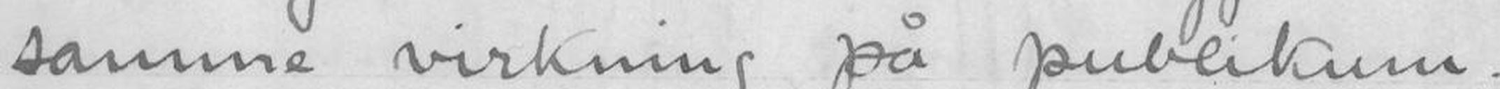samme virkning på publikum.
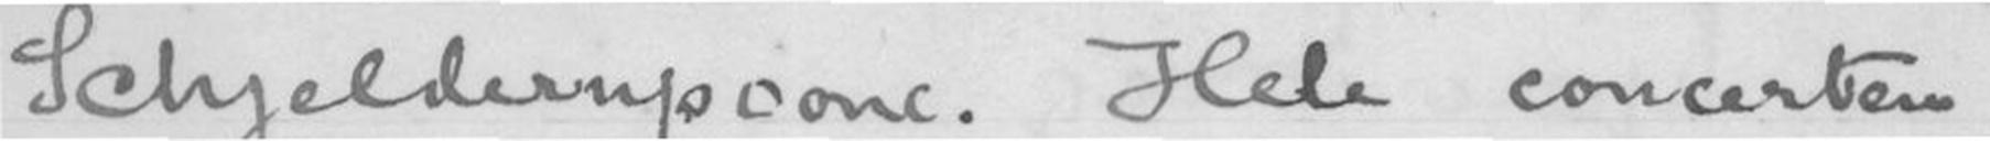Schjelderupconc. Hele concerten
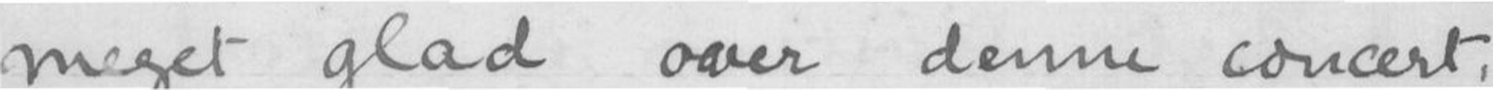meget glad over denne concert,
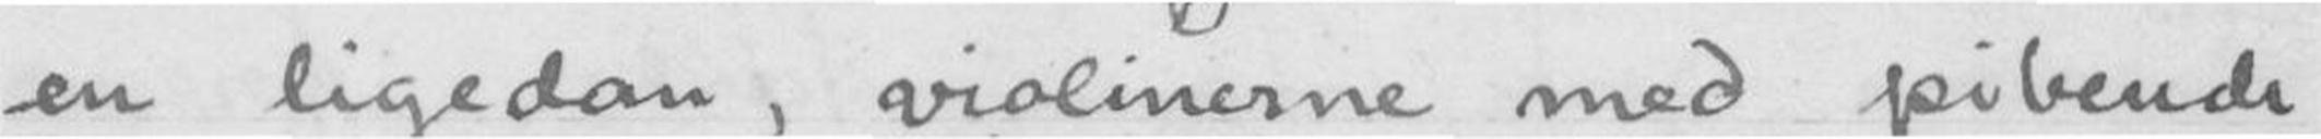en ligedan, violinerne med pibende
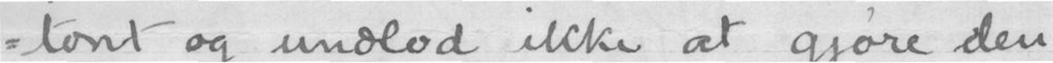tont og undlod ikke at gjøre den
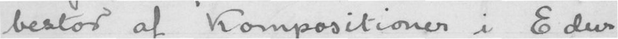bestod af Kompositioner i E dur
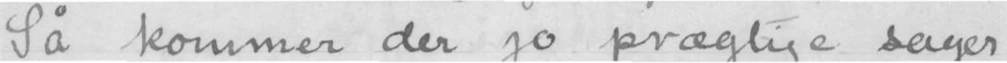Så kommer der jo prægtige sager
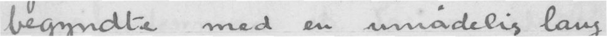begyndte med en umådelig lang
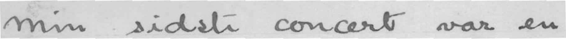Min sidste concert var en
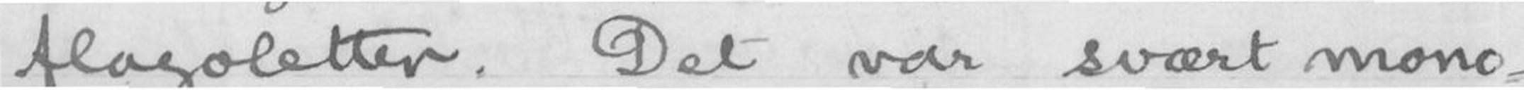flagoletter. Det var svært mono-
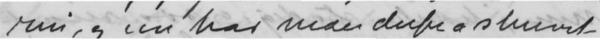rin, og nu har man derfor a skrevet
